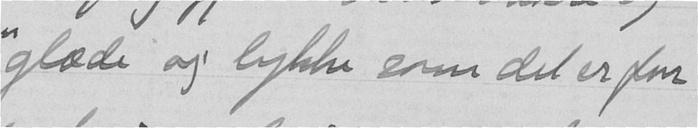glæde og lykke som det er for
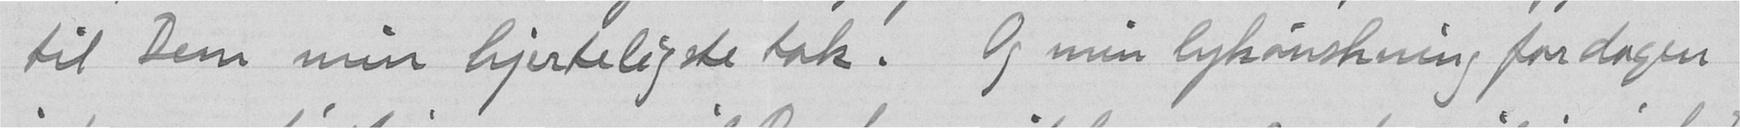til Dem min hjerteligste tak. Og min lykønskning for dagen
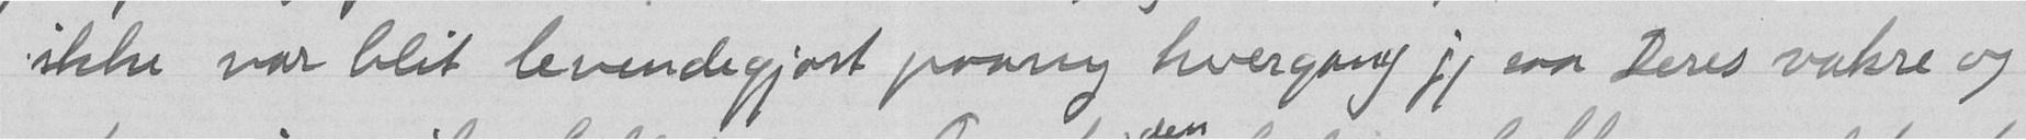ikke var blit levendegjort paany hvergang jeg saa Deres vakre og
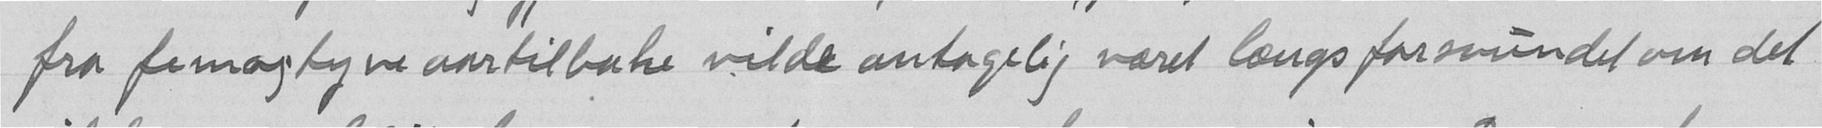fra femogtyve aar tilbake vilde antagelig været længs forsvundet om det
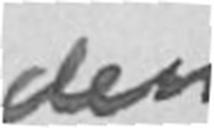den
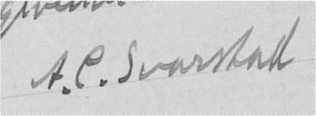A. C. Svarstad
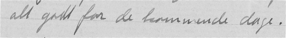alt godt for de kommende dage.
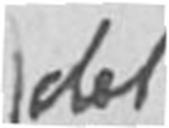det
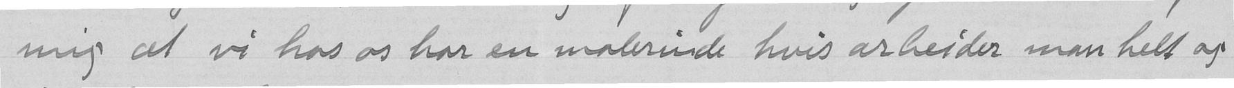mig at vi hos os har en malerinde hvis arbeider man helt og
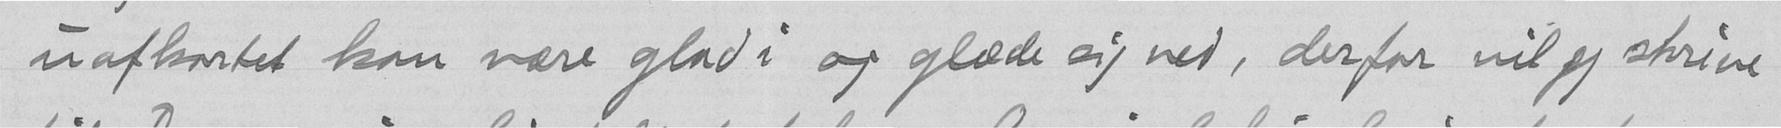uafkortet kan være glad i og glæde sig med, derfor vil jeg skrive
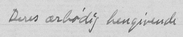Deres ærbødig hengivende
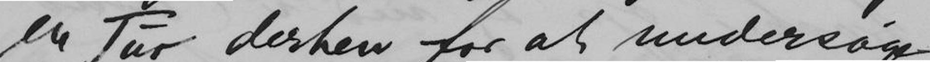en Tur derhen for at undersøge
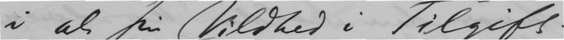i al sin Vildhed i Tilgift.
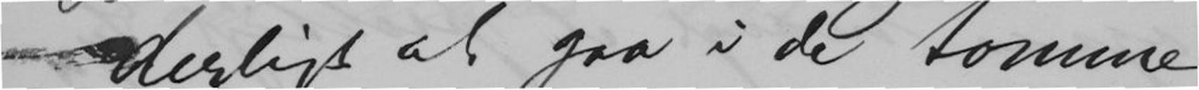derligt at gaa i de tomme
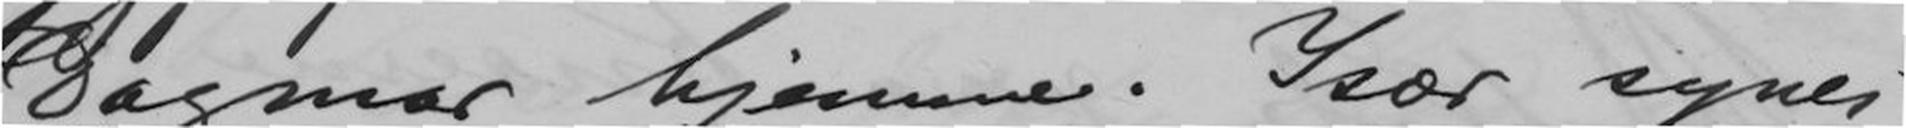Dagmar hjemme. Især synes
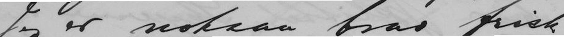Jeg er noksaa bra frisk
